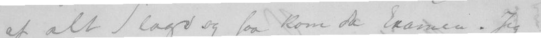af alt Slags og saa kom da Examen. Jeg
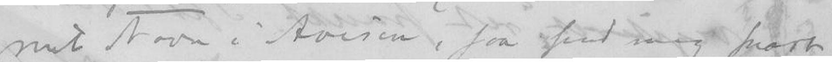mit Navn i Avisen, saa send mig snart
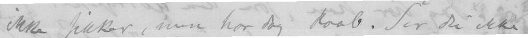ikke sikker, men har dog Haab. Ser Du ikke
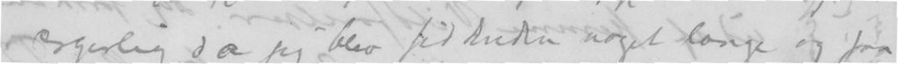ærgelig da jeg blev siddende noget længe og saa
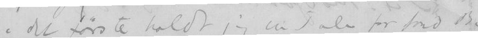i det første hold jeg en Tale for fred Be-
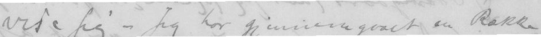vise sig. Jeg har gjennemgaaet en Række
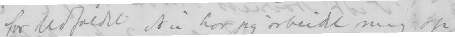for Udfaldet. Nu har jeg arbeide mig op
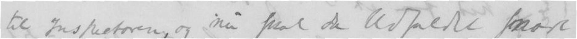til Indspectøren, og nu skal da Udfalde snart
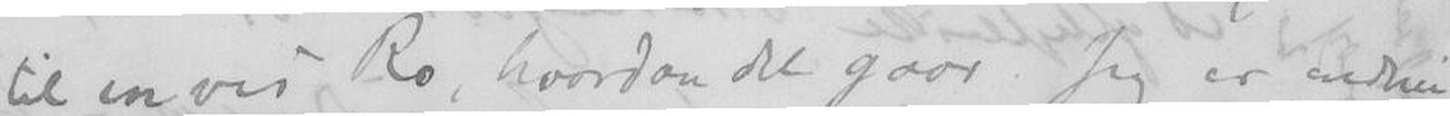til en vis Ro, hvordan det gaar. Jeg er endnu
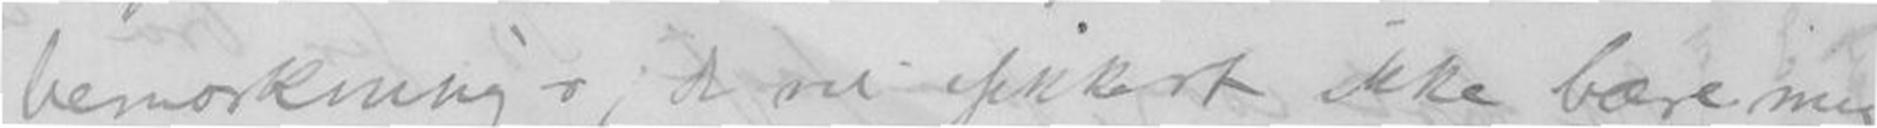bemærkninger; de vil sikkert ikke bære
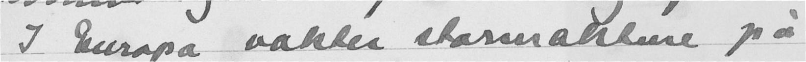I Europa vokter stormaktene på
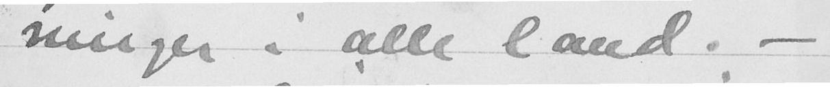ninger i alle land. -
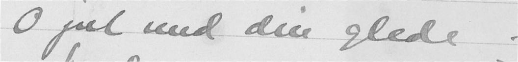O jul med din glede og
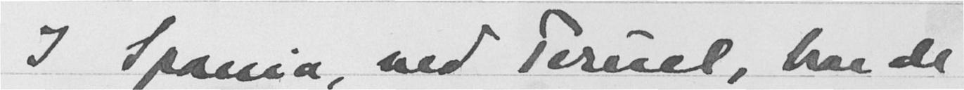I Spania, ved Tesuel, har de
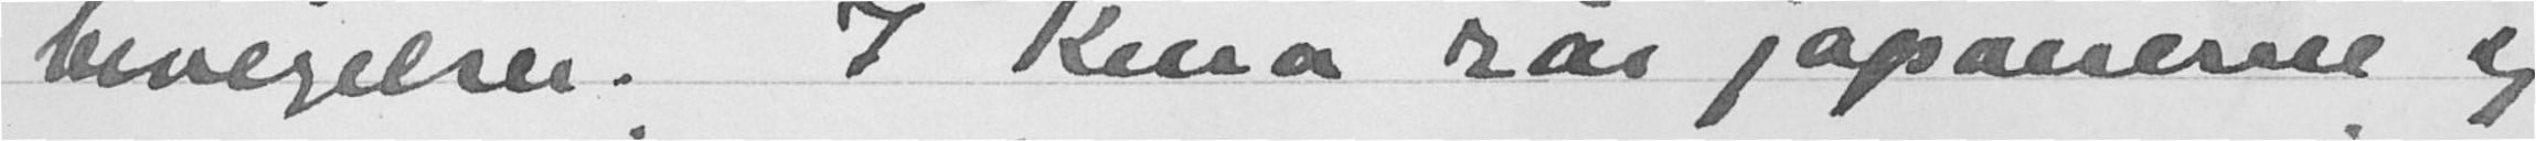bevegelser. I Kina rår japanerne sig
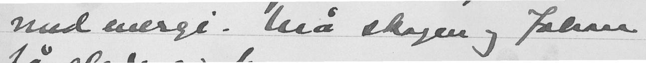med energi. Må skogen og Johan
